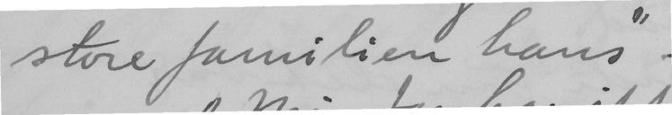store familien hans" –
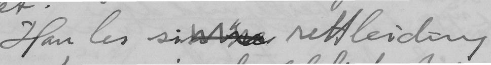Han les sin rettleiding
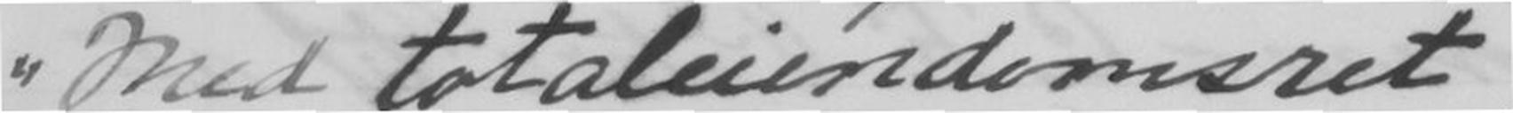"Med totaleiendomsret
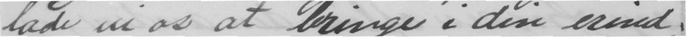lade vi os at bringe i din erind
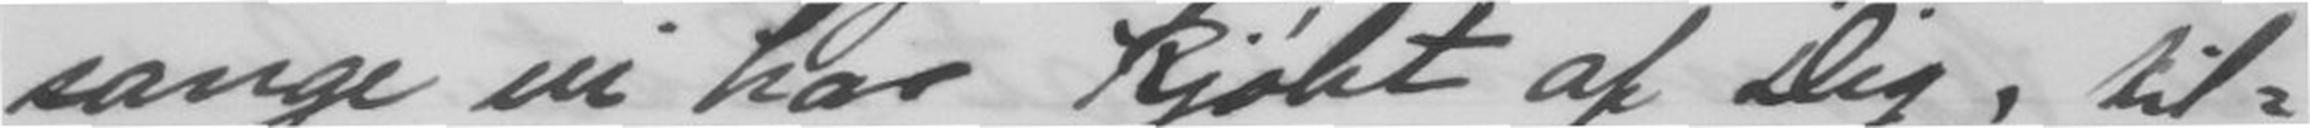sange vi har kjøbt af Dig, til-
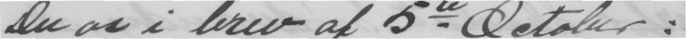Du os i brev af 5te October:
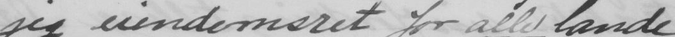jeg eiendomsret for alle lande
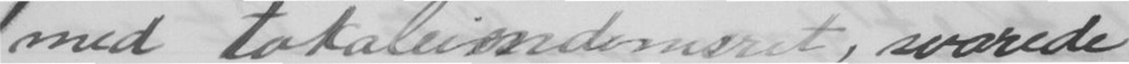med totaleiendomsret, svarede
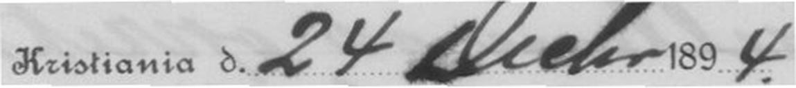Kristiania d. 24 Decbr 1894.
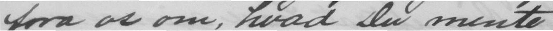fora os om, hvad Du mente
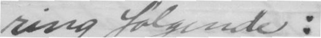ring følgende:
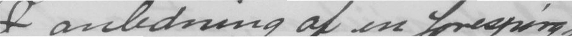I anledning af en forespørgsel
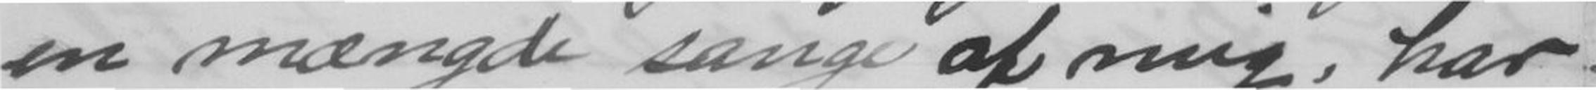en mængde sange af mig, har
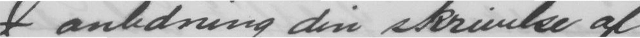I anledning din skrivelse
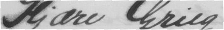Kjære Grieg
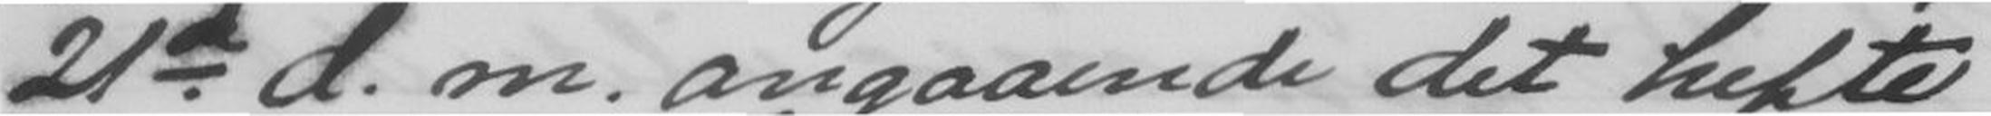af 21de d. m. angaaende det hefte
